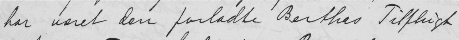har været den forladte Berthas Tilflugt
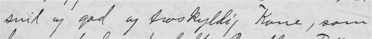snil og god og troskyldig Kone, som
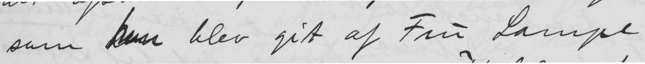som kun blev git af Fru Lampe
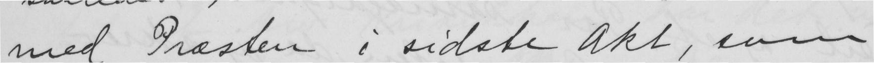med Præsten i sidste Akt, som
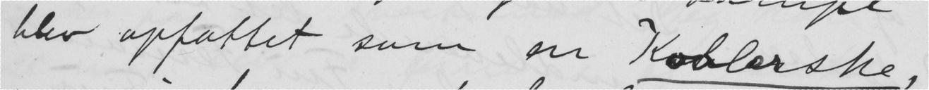blev opfattet som en Koblerske,
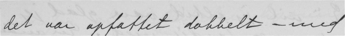det var opfattet dobbelt - med
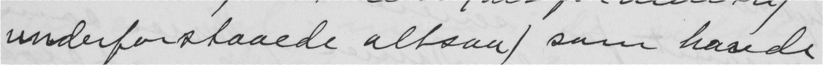underforstaaede altsaa) som havde
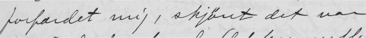forfærdet mig, skjønt det var
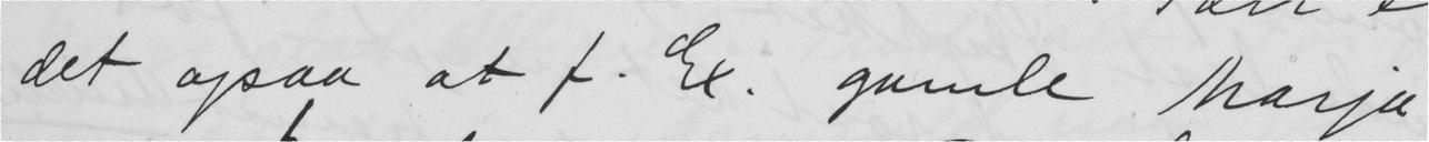det ogsaa at f. Ex. gamle Marja
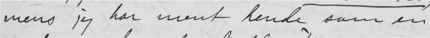mens jeg har ment hende som en
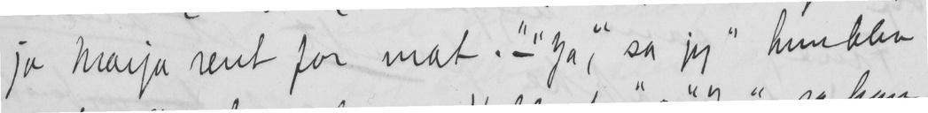jo Marja rent for mat." - "Ja", sa jeg "hun blev
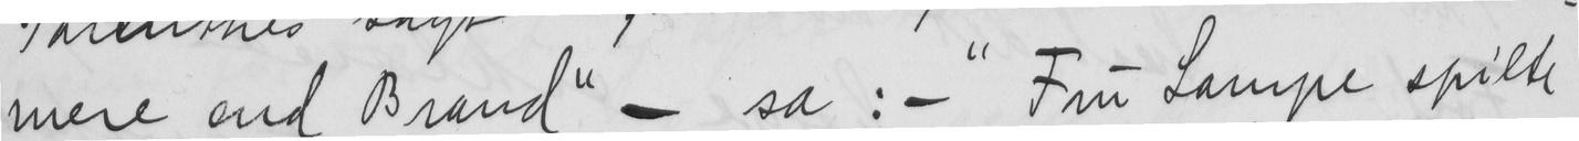mere end Brand" - sa: - "Fru Lampe spilte
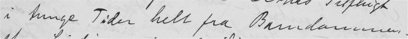i tunge Tider helt fra Barndommen.
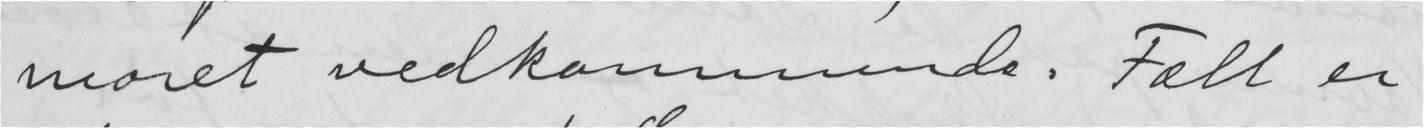moret vedkommende. Fælt er
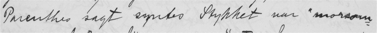Parenthes sagt syntes Stykket var "morsom-
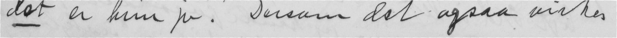det er hun jo". Dersom det ogsaa virker
